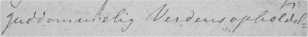guddommelig Verdens opholdel-
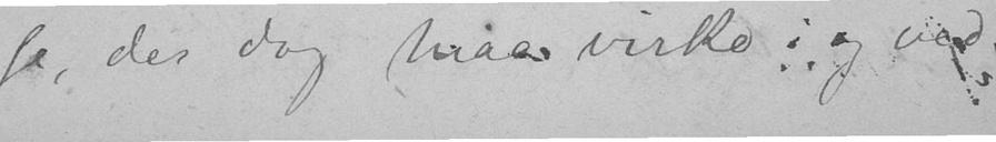se, der dog maa virke i og ved
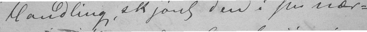Handling, skjønt den i sin nær-
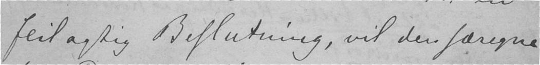feilagtig Beslutning, vil den særegne
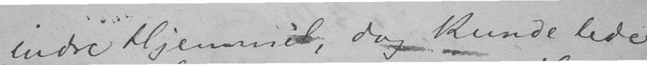indre Hjemmel, dog kunde lede
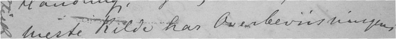meste Kilde har Overbeviisningens
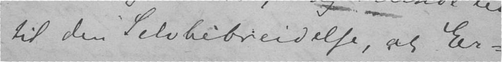til den Selvbebreidelse, at Er-
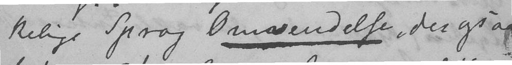kelige Sprog Omvendelse, der ogsaa
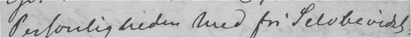Personligheden med fri Selvbevidst-
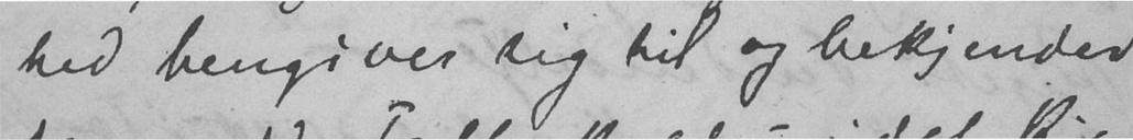hed hengiver sig til og bekjender
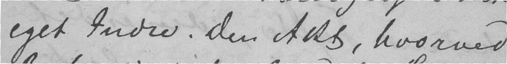eget Indre. Den Akt, hvorved
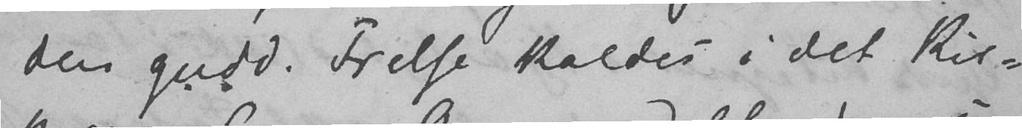den gudd. Frelse kaldes i det Kir-
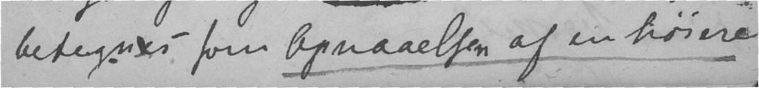betegnes som Opnaaelsen af en høiere
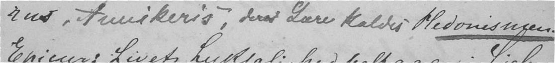sus Ankers, deres Lære kaldes Hedonismen.
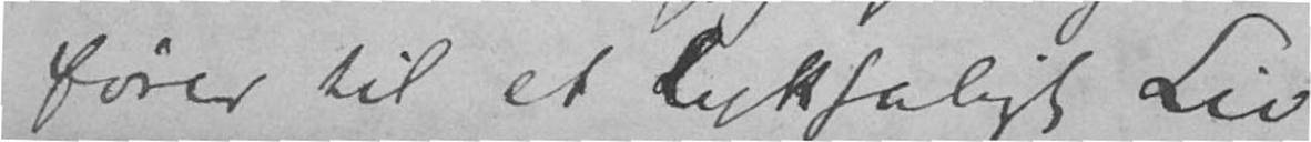fører til et lyksalig Liv
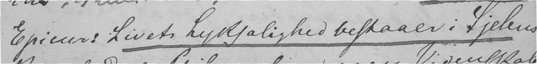Epicur: Livets Lyksalighed bestaaer i Sjelens
